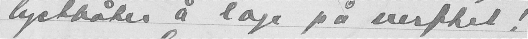lystbåter å lage på verftet.
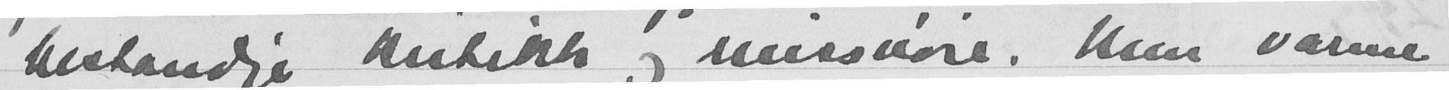bestandige kritikk og misnøie. Men varme
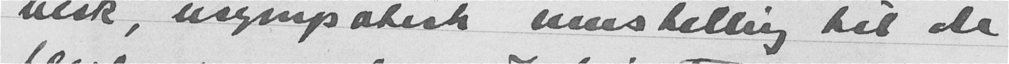besk, usympatisk innstilling til de
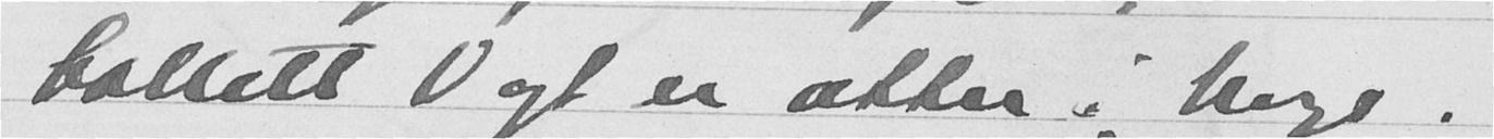Collett Vogt er atter i Norge.
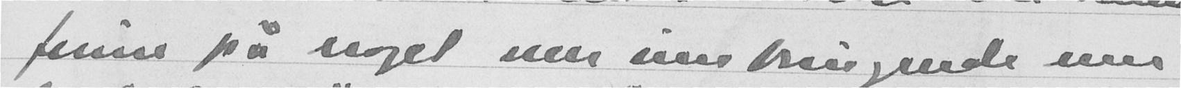finne på noget mer innsmigrende enn
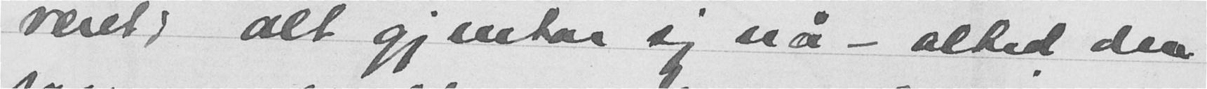været, alt gjentar sig nå - altid den
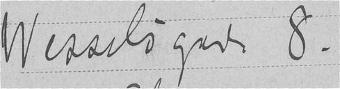Wesselsgade 8.
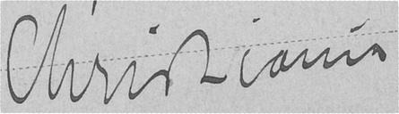Christiania
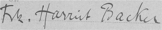Frk. Harriet Backer
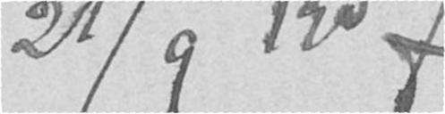21/9 1907
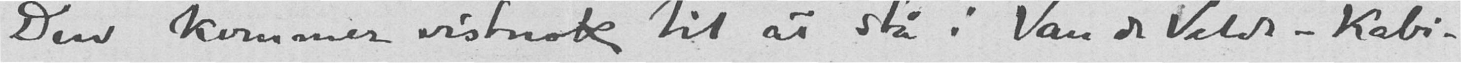Den kommer vistnok til at stå i Van der Velde-kabi-
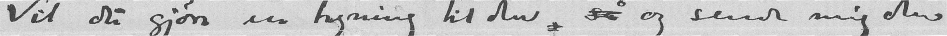Vil du gjøre en tegning til den, så og sende mig den
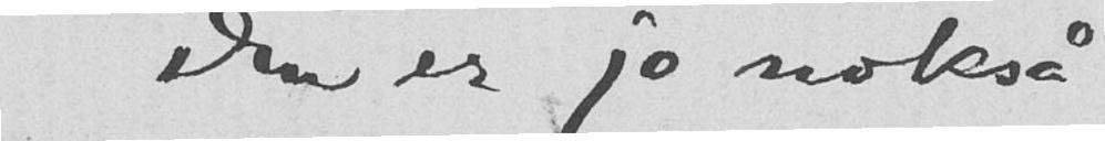Den er jo nokså
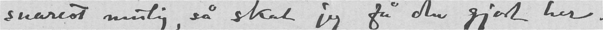snarest mulig, så skal jeg få den gjort her.
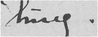tung.
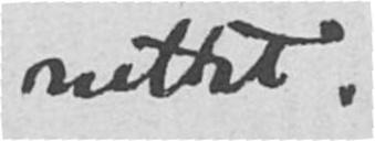nettet.
